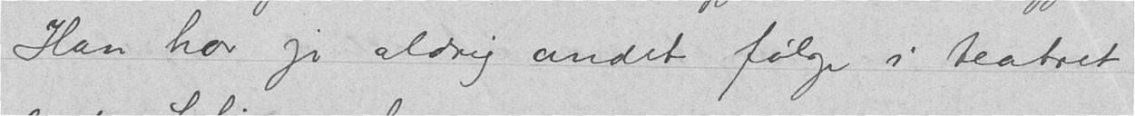Han har jo aldrig andet følge i teatret
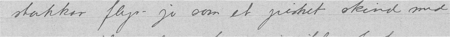stakkar flyr jo som et pisket skind med
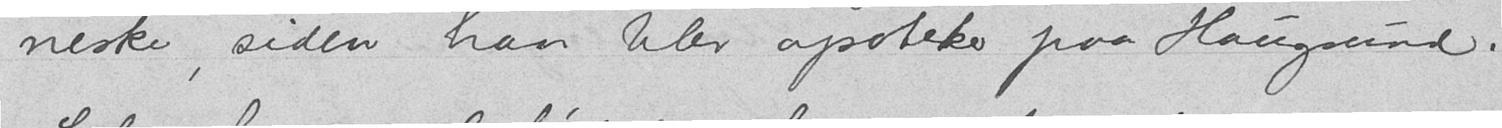neske, siden han blev apoteker paa Haugsund.
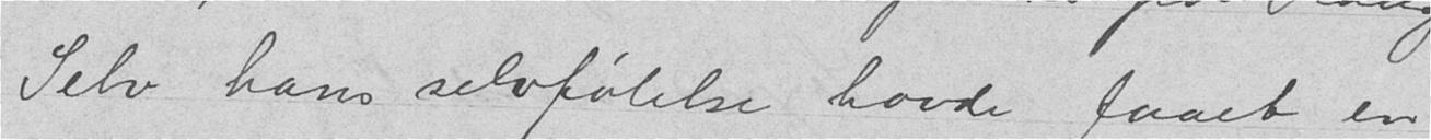Selv hans selvfølelse havde faaet en
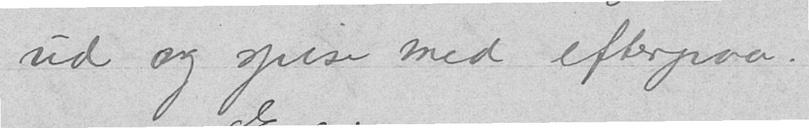ud og spise med efterpaa.
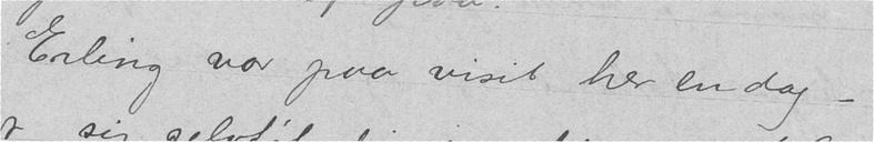Erling var paa visit her en dag –
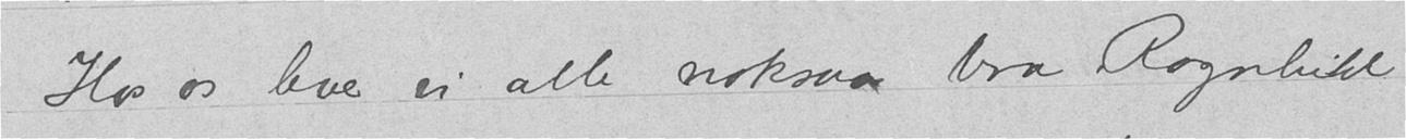Hos os lever vi alle noksaa bra. Ragnhild
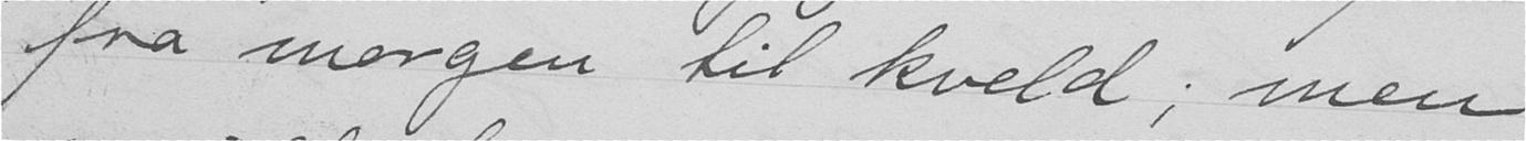fra morgen til kveld; men
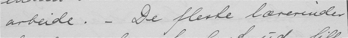arbeide. - De fleste lærerinder
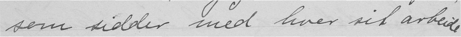som sidder med hver sit arbeide -
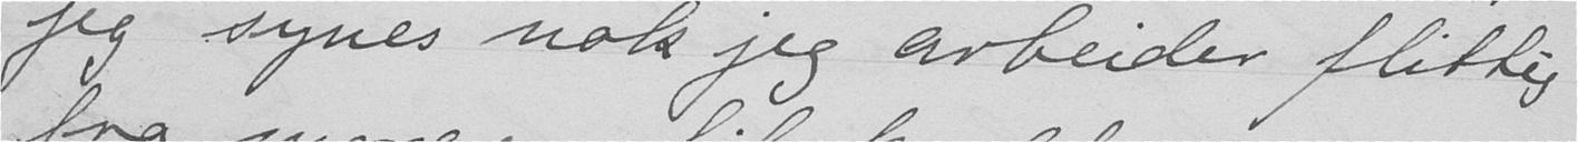jeg synes nok jeg arbeider flittig
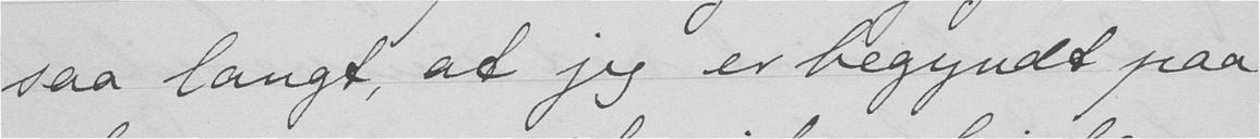saa langt, at jeg er begyndt paa
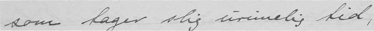som tager slig urimelig tid;
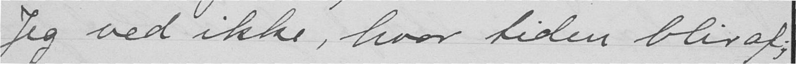Jeg ved ikke, hvor tiden blir af;
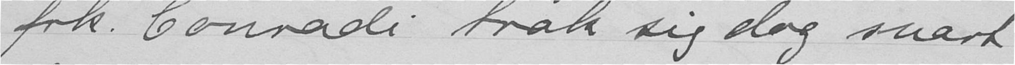frk. Conradi trak sig dog snart
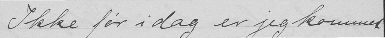Ikke før idag er jeg kommet
